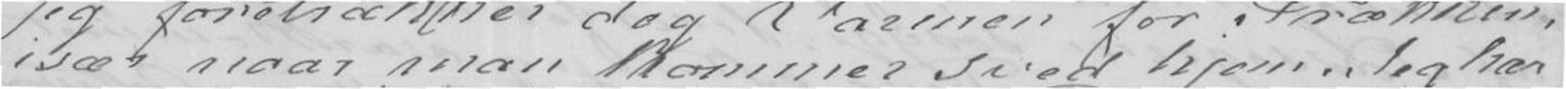især naar man kommer sved hjem. Jeg har
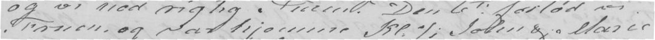Fruen, og var hjemme Kl.7; John og Marie
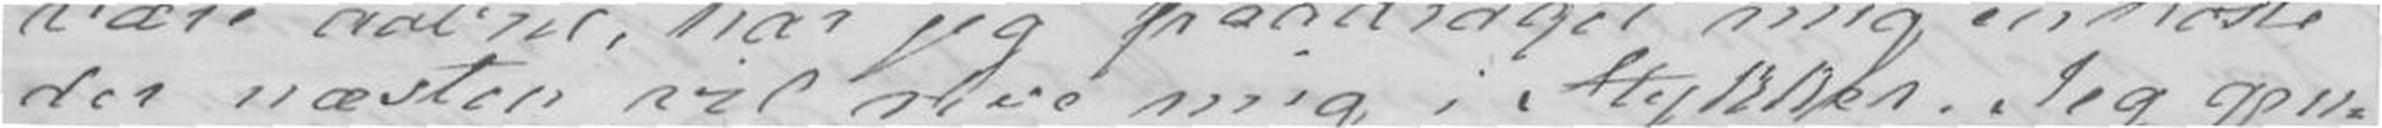der næsten vil rive mig i Stykker. Jeg gru-
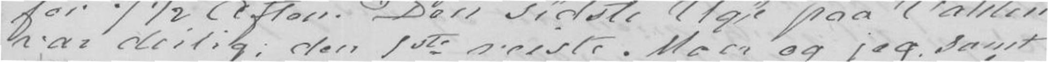var deilig; den 1ste reiste Moer og jeg samt
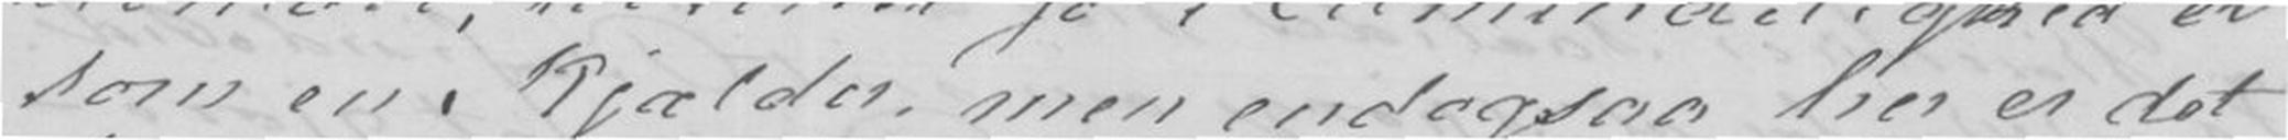som en Kjælder, men endogsaa her er det
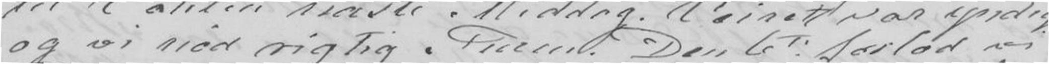og vi nød rigtig Turen. Den 6te forlod vi
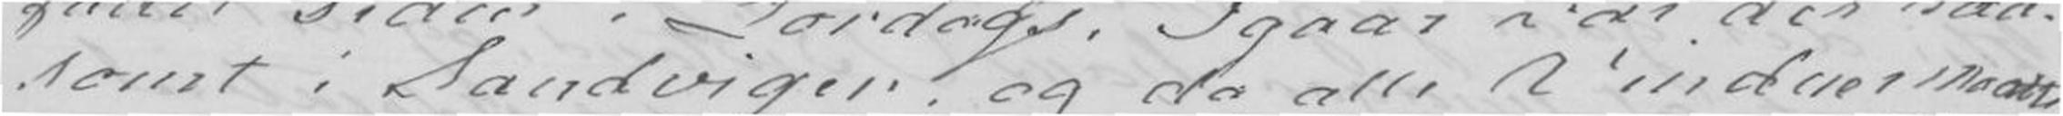somt i Sandvigen, og da alle Vinduer maatte
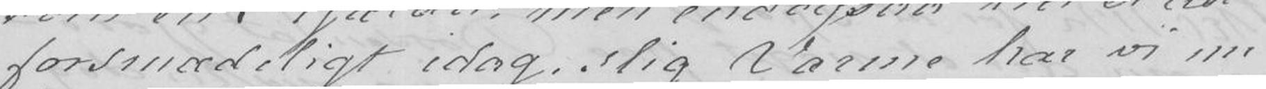forsmædeligt idag, slig Varme har vi nu
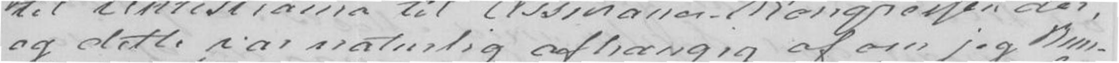og dette var naturlig afhængig af om jeg kun-
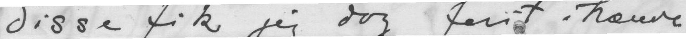disse fik jeg dog først i hænde
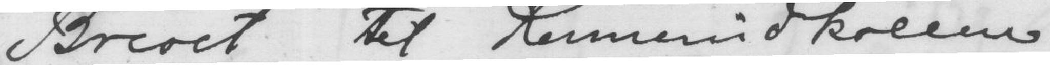Brevet til Kumerudkollen,
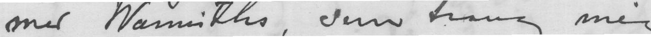med Warmuths, som tvang mig
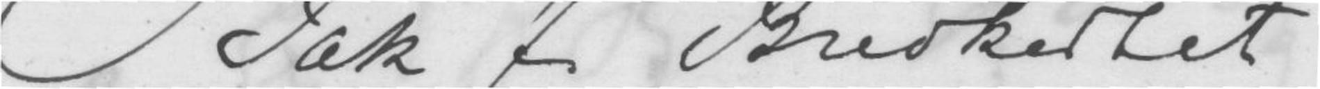Tak for Brevkortet og
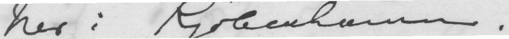her i Kjøbenhavn.-
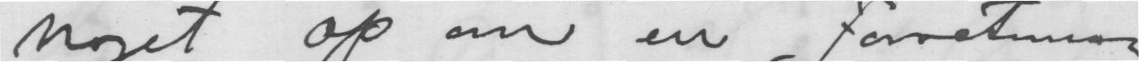noget op om en forretning
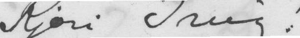Kjære Grieg!
